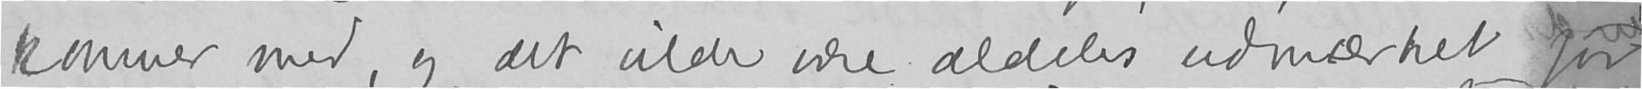kommer med, og det vilde være aldeles udmærket for
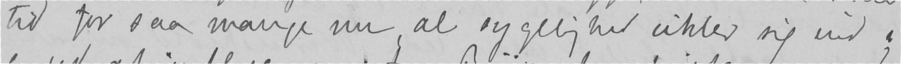tid for saa mange nu, al sygelighed vikler sig ind i
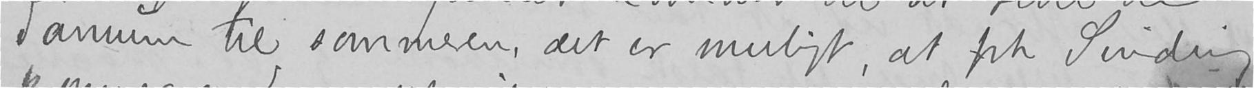Tannum til sommeren, det er muligt at frk Sinding
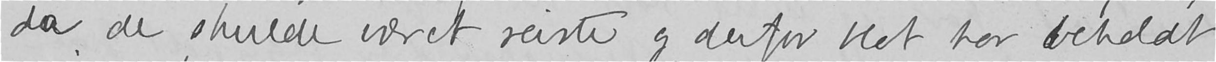da de skulde været reiste og derfor blot har beholdt
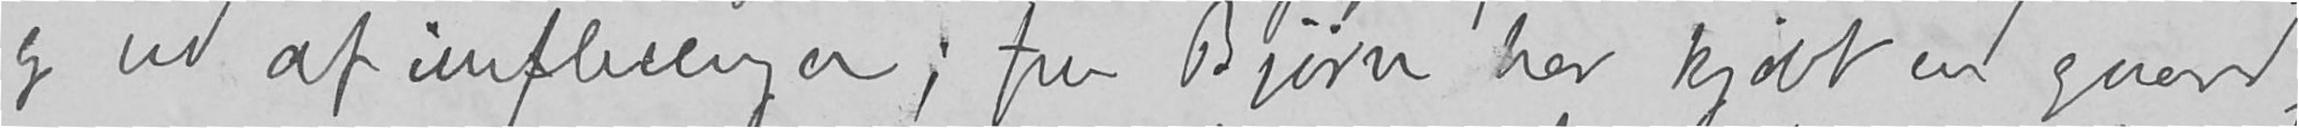og ud af influenzen; fru Bjørn har kjøbt en gaard
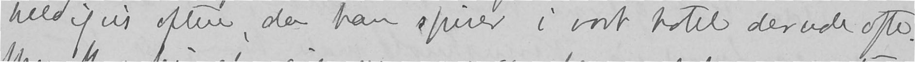heldigvis oftere, da han spiser i vort hotel derude ofte.
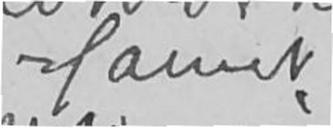Harriet
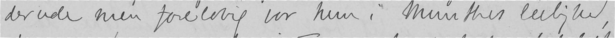derude men foreløbig bor hun i Munthes leilighed,
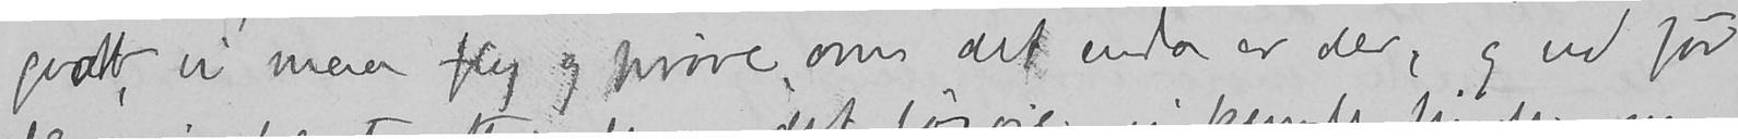gaat, vi maa fly og prøve, om det enda er der, og ud for
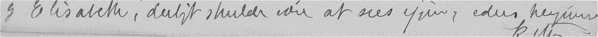og Elisabeth, deiligt skulde være at sees igjen, eders hengivne
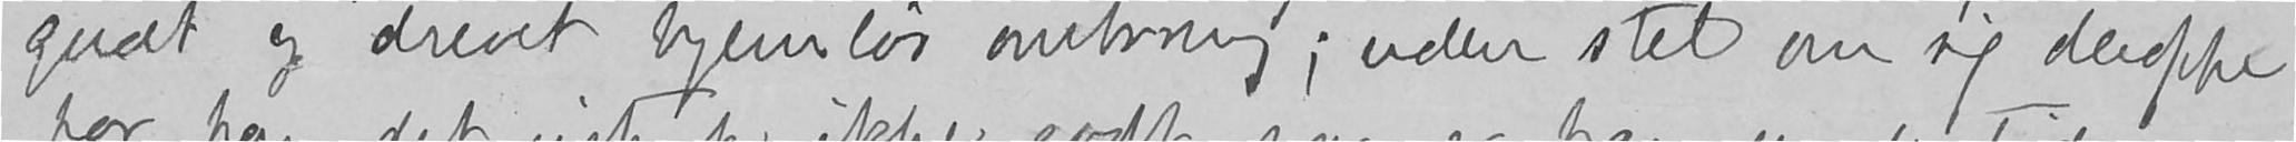gaat og drevet hjemløs omkring; uden stel om sig deroppe
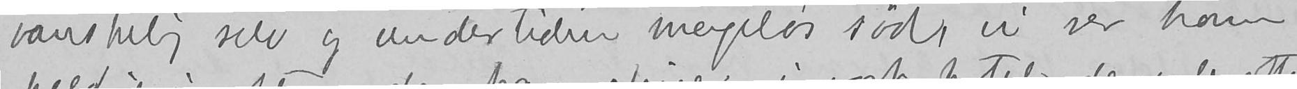vanskelig selv og undertiden mageløs sød, vi ser ham
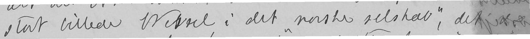stort billede Wessel i det "norske selskab", det ser
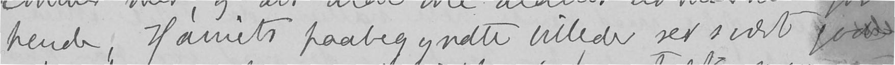hende, Harriets paabegyndte billede ser svært godt
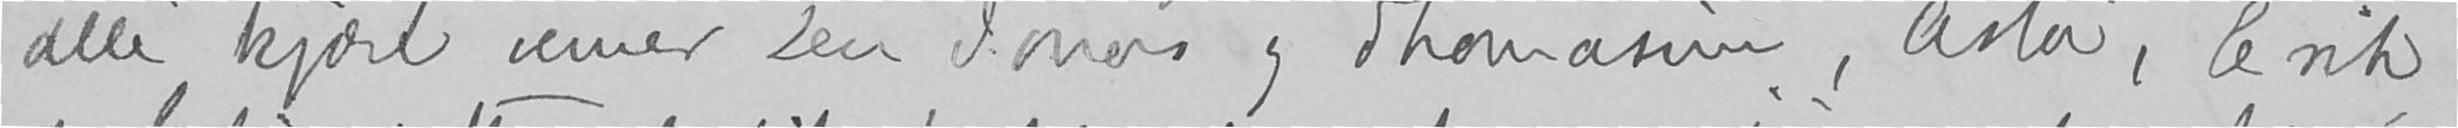alle kjære venner Du Jonas og Thomasine, Asta, Erik
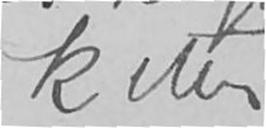Kitty
